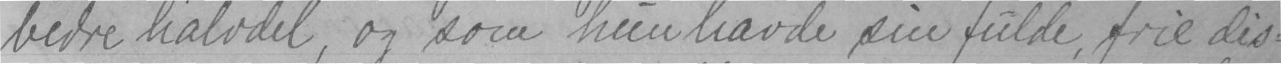bedre halvdel, og som hun havde sin fulde, frie dis-
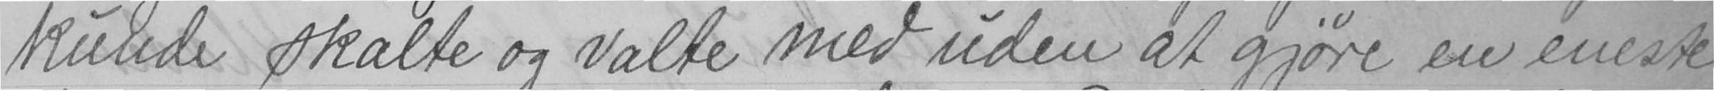kunde skalte og valte med uden at gjøre en eneste
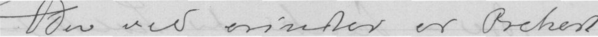Du vel erindrer er Orchest-
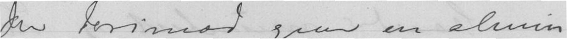Du derimod give en almin
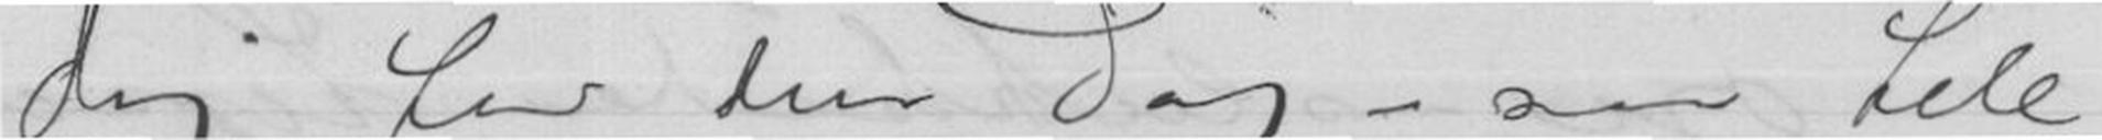Dig for den Dag - saa tele-
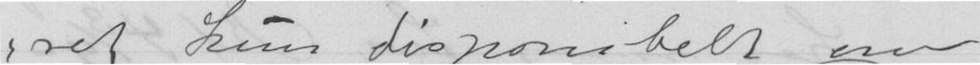ret kun disponibelt om
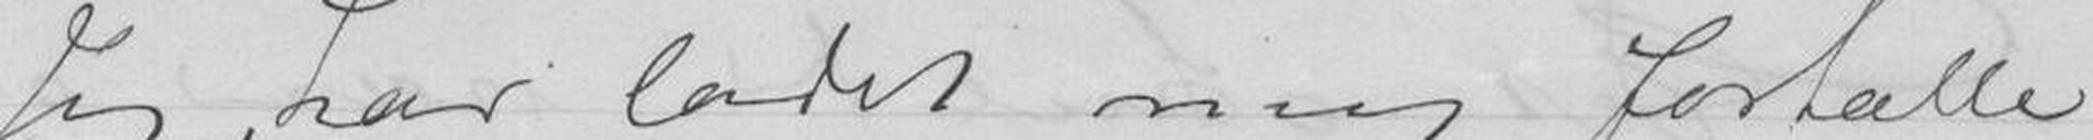Jeg har ladet mig fortælle
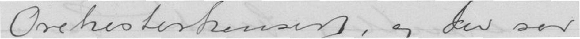Orchesterkonsert, og Du ser
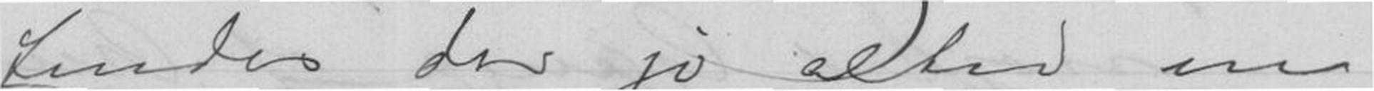findes der jo altid en
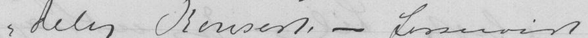delig Konsert - forsaavidt
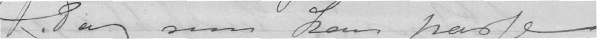Dag som kan passe
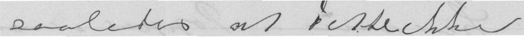saaledes at Dette ikke
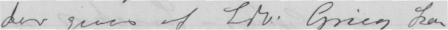der gives af Edv. Grieg kan
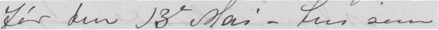før den 13e Mai - thi som
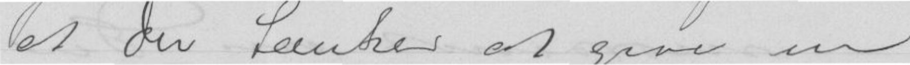at Du tænker at give en
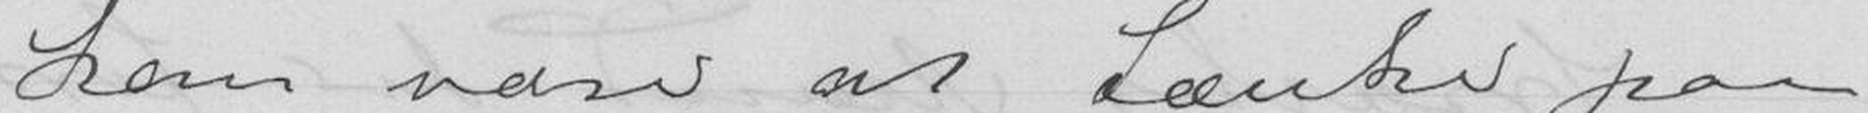kan være at tænke paa
